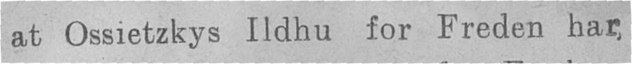at Ossietzkys Ildhu for Freden har
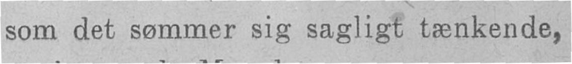som det sømmer sig sagligt tænkende,
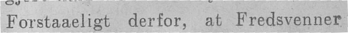Forstaaeligt derfor, at Fredsvenner
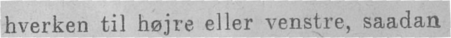hverken til højre eller venstre, saadan
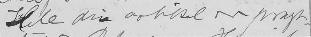Hele din artikel er pragt-
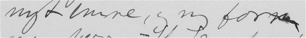nyt emne, og ny form
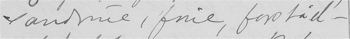sandrue, fine, forståel-
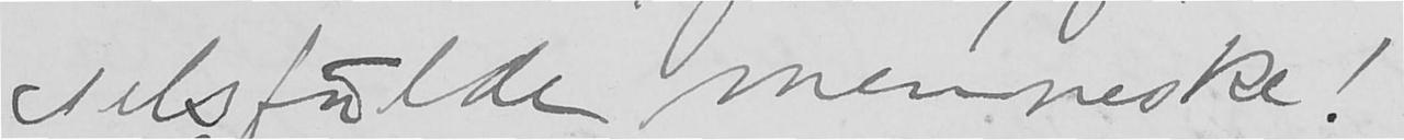selsfulde menneske!
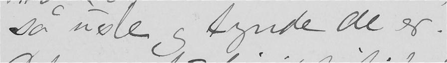så usle og tynde de er.
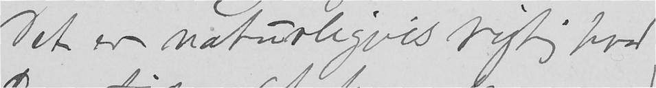Det er naturligvis rigtig hvad
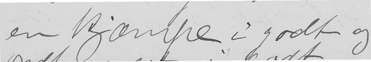en kjæmpe i godt og
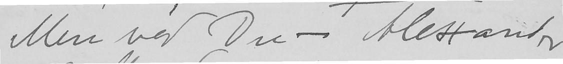Men véd Du - Alexander
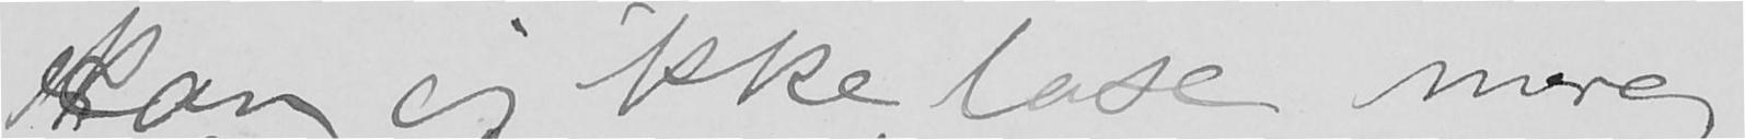kan jeg ikke læse mere,
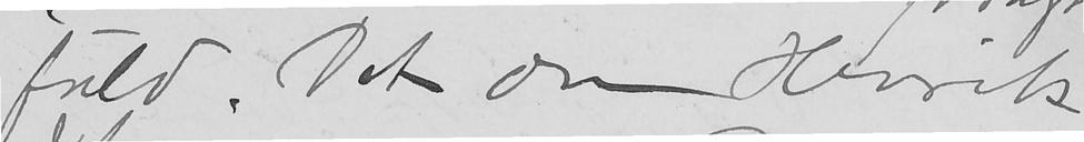fuld. Det om Henrik
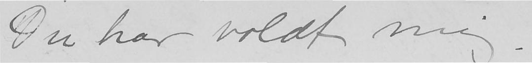Du har voldt mig.
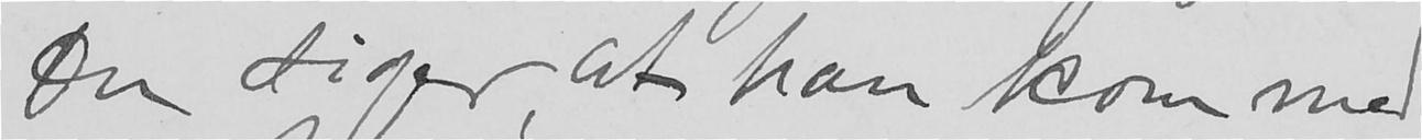Du siger, at han kom med
